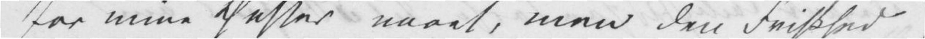for mine Ønsker naaet, men den Friskhed
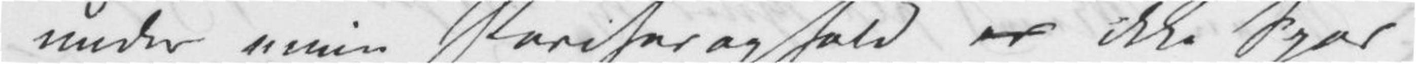under mine Pariserophold er ikke Spor
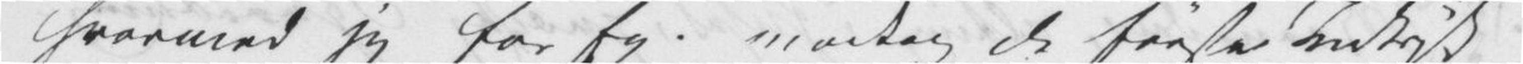hvormed jeg for Ex. modtog de første Indtryk
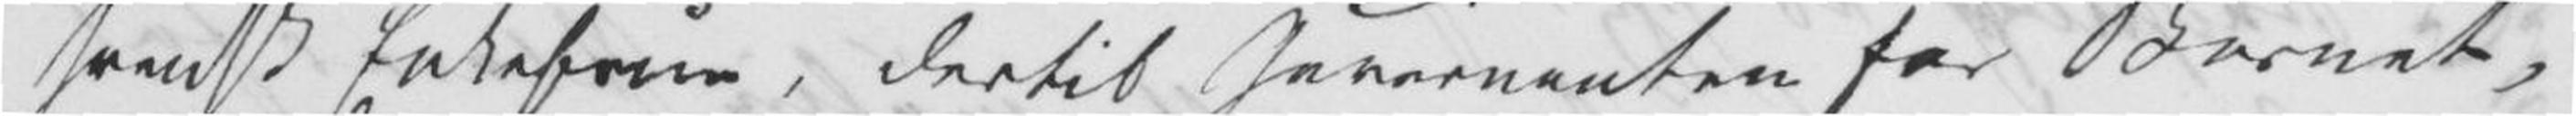svensk Enkefrue, dertil Guvernanten for Barnet,
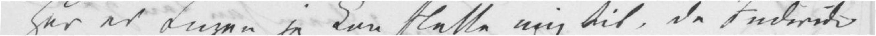Her er Ingen jeg kan slutte mig til, de Individer
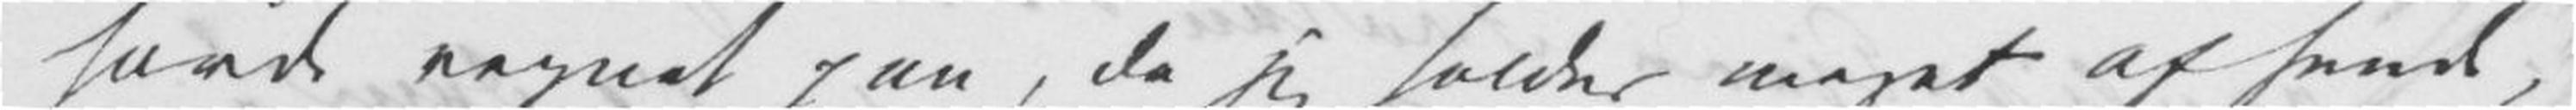havde regnet paa, da jeg holder meget af hende,
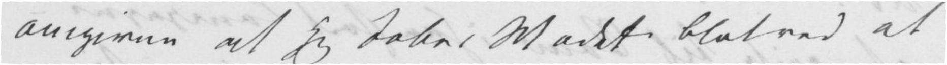omgivne at jeg taber Modet blot ved at
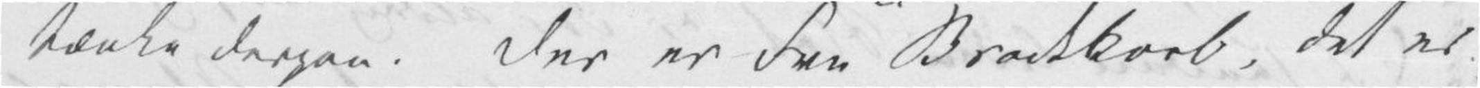tænke derpaa. Der er Fru Brodtkorb, det er
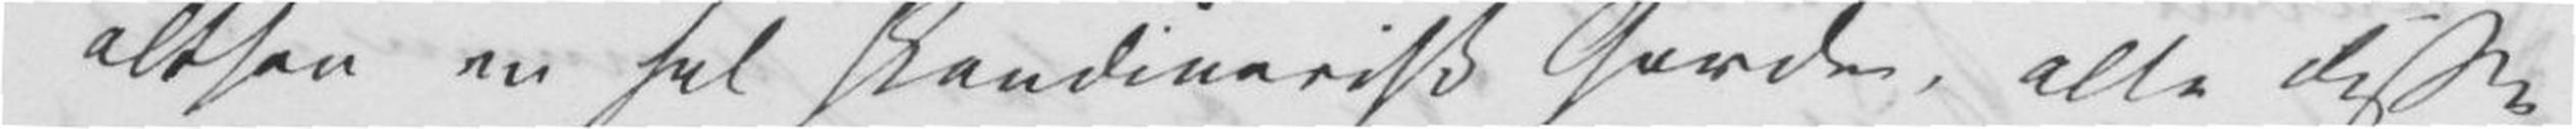altsaa en hel skandinavisk Garde, alle disse
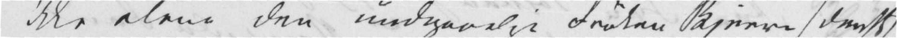ikke alene den undgaaelige Frøken Bjerre/dansk
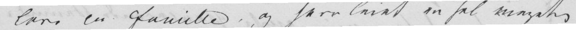lave en Familie, og have leiet en hel meget
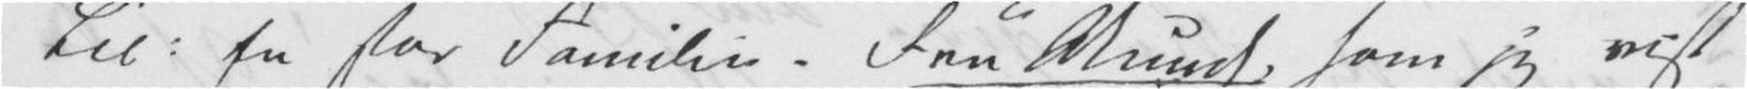Lie: En stor Familie. Fru Munch, som jeg vist
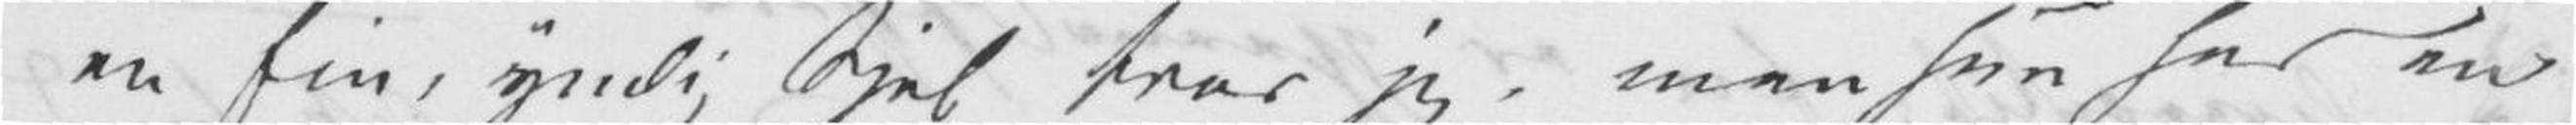en fin, yndig Sjel tror jeg, men hun har en
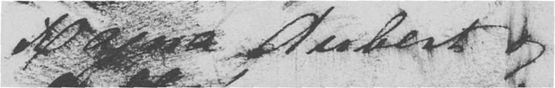Ragna Aubert og
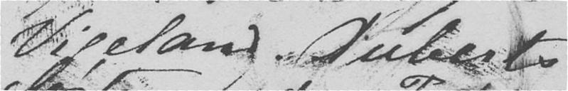Vigeland. Auberts
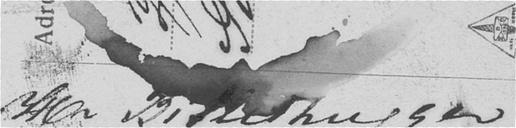Hr Billedhugger
